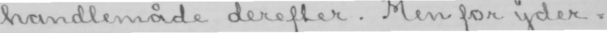handlemåde derefter. Men for yder-
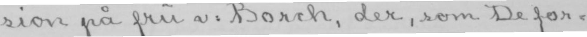sion på fru v: Borch, der, som De for-
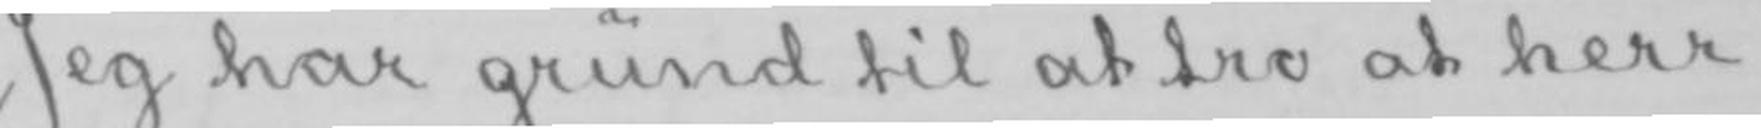Jeg har grund til at tro at herr
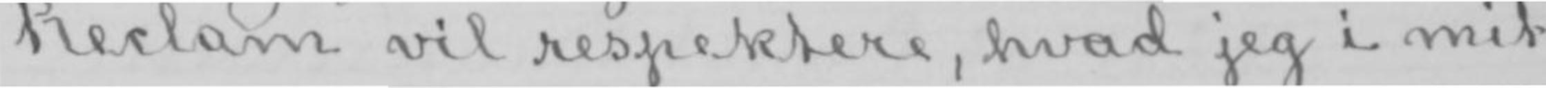Reclam vil respektere, hvad jeg i mit
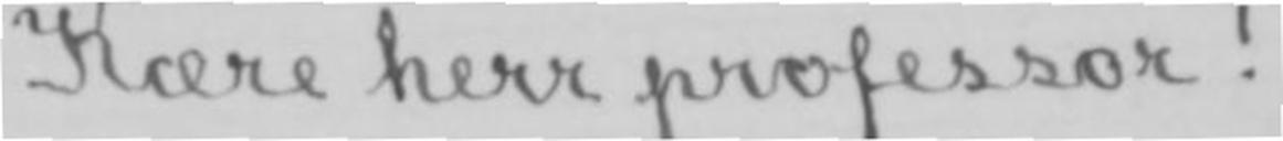Kære herr professor!
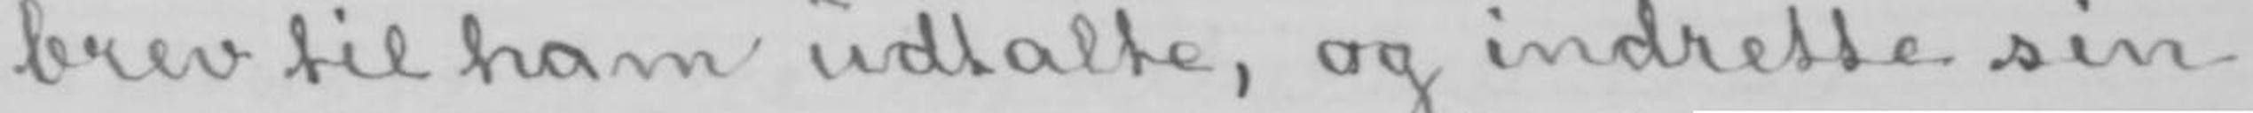brev til ham udtalte, og indrette sin
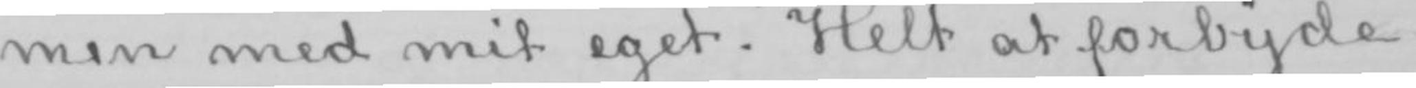men med mit eget. Helt at forbyde
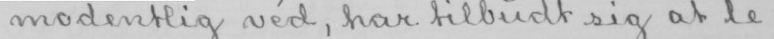modentlig véd, har tilbudt sig at le-
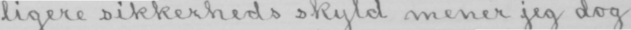ligere sikkerheds skyld mener jeg dog
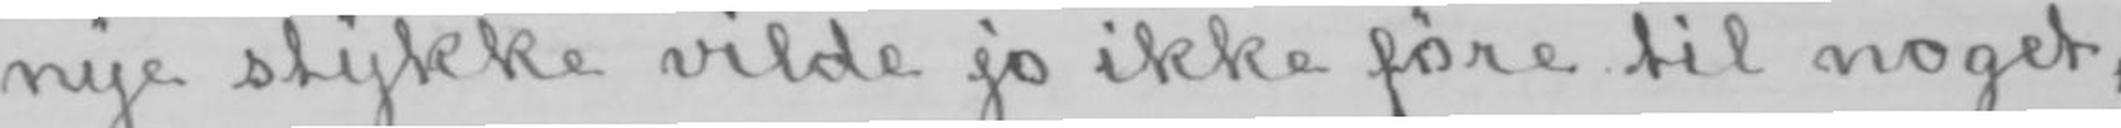nye stykke vilde jo ikke føre til noget,
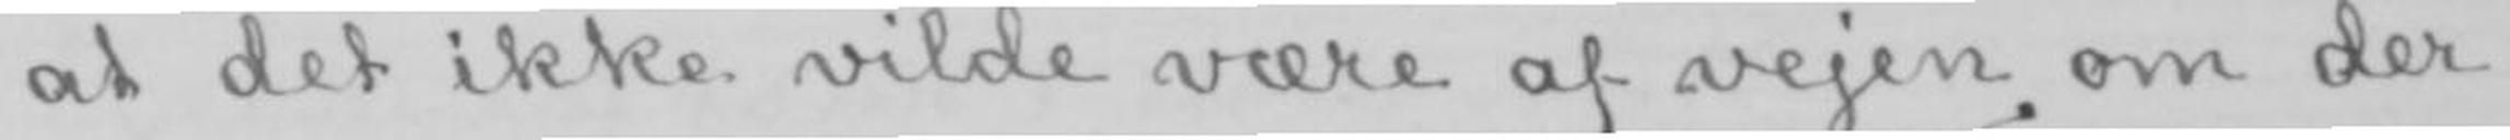at det ikke vilde være af vejen om der
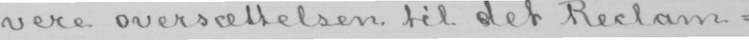vere oversættelsen til det Reclam-
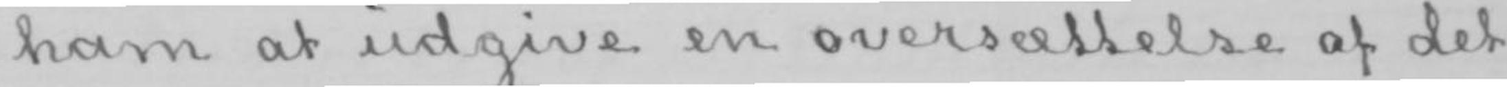ham at udgive en oversættelse af det
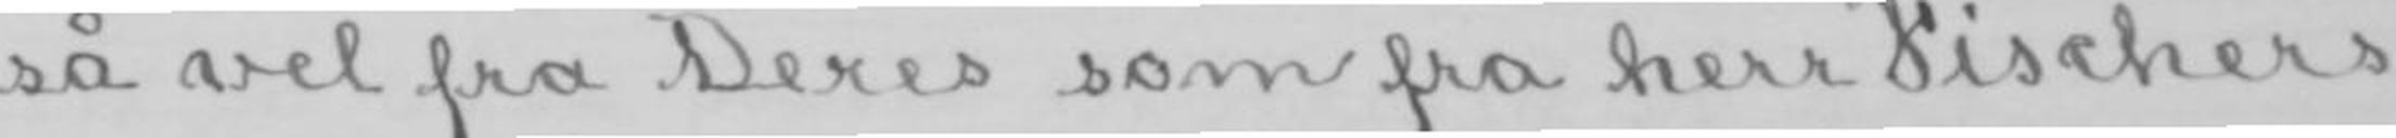så vel fra Deres som fra herr Fischers
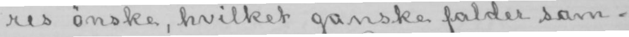res ønske, hvilket ganske falder sam-
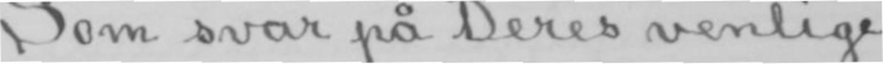Som svar på Deres venlige
